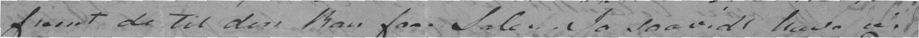fremt de til den kan faae Salen. Ja saavidt have vi
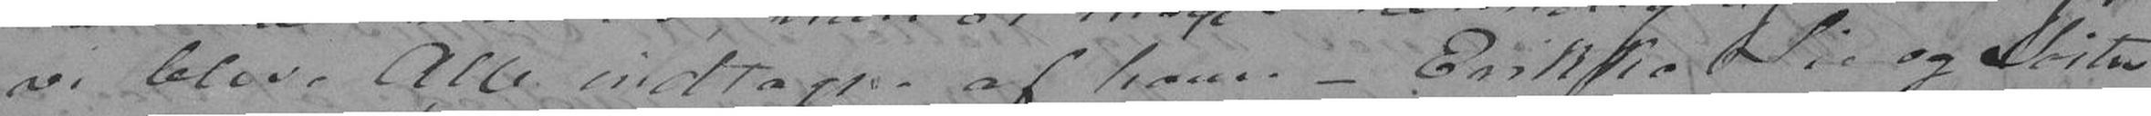vi blev Alle indtagne af ham. - Erikka Lie og Søster
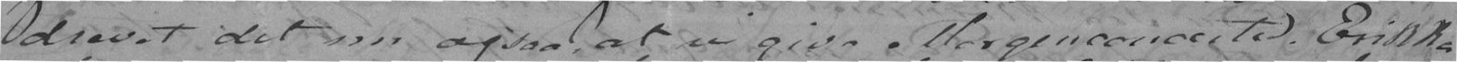drevet det nu ogsaa, at give Morgenconcert. Erikka
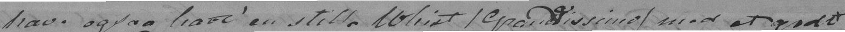have ogsaa havt en stille Whist/GrandVissimo/ med et godt
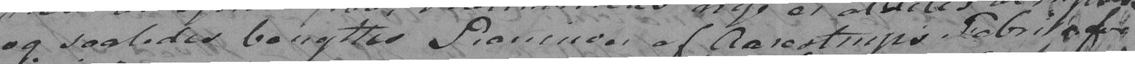og saaledes benyttes Pianenoer af Aarestrups Fabrik, for-
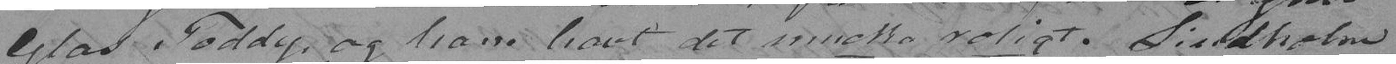Glas Toddy, og have havt det mucke roligt. Lindholm
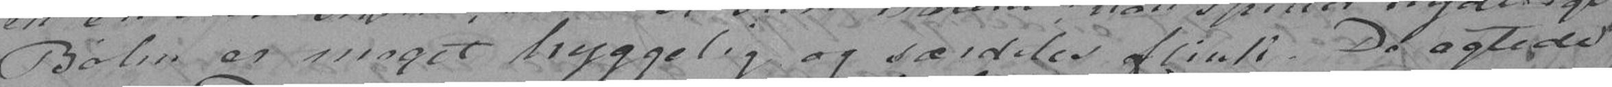Bøhn er meget hyggelig og særdeles flink. De agtede
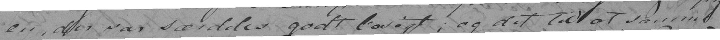en, der var særdeles godt besøgt; og det til at samme
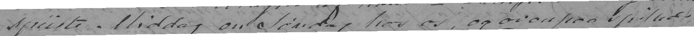spiiste Middag en Søndag hos os, og ovenpaa spillede
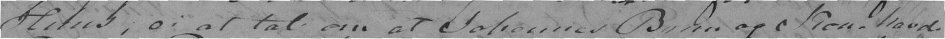Huus; ei at tale om at Johannes Brun og Kone havde
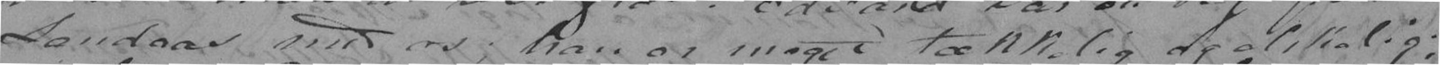Landaas med os; han er meget tækkelig og alikelig,
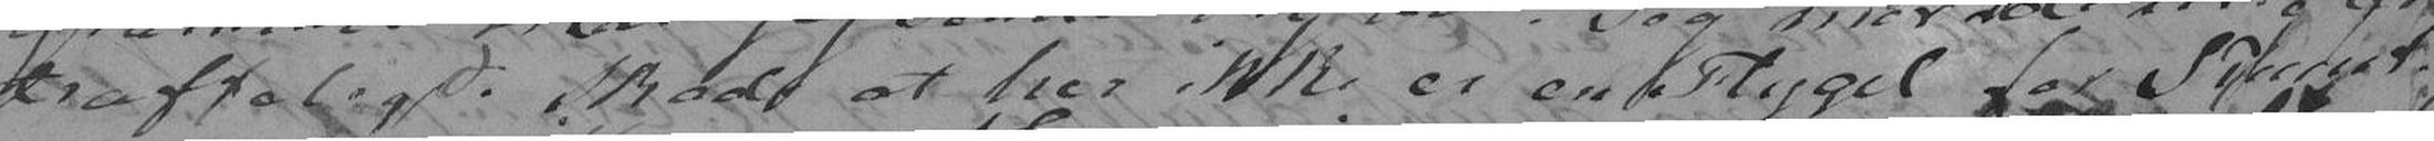træffeligt. Skade at her ikke er en Flygel for Kunst-
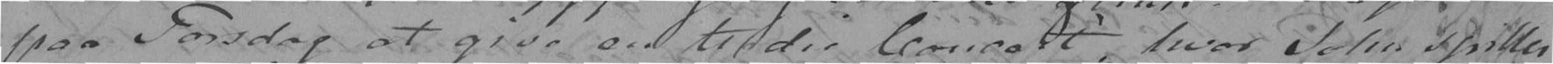paa Torsdag at give en tredie Concert, hvor John spiller
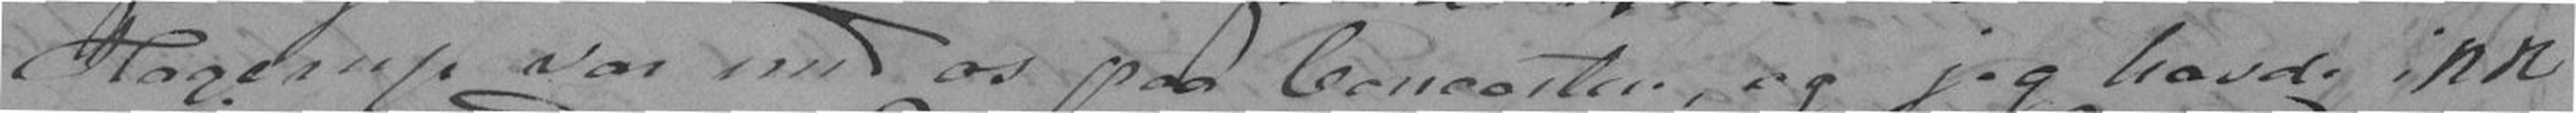Hagerup var med os paa Concerten, og jeg havde ikke
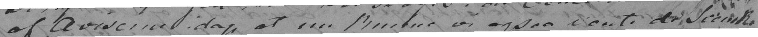af Aviserne idag at nu kunne vi ogsaa vente dr Svenske
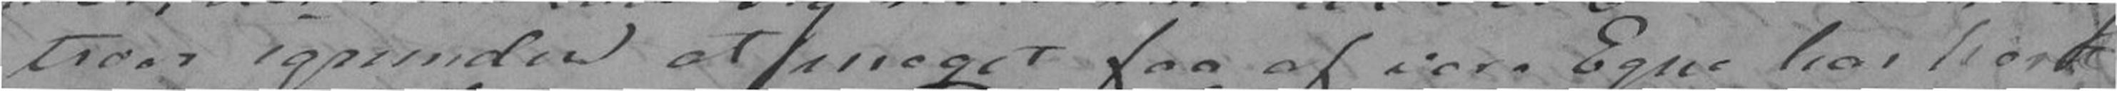troer igrunden at meget faa af vore Egne har hørt
